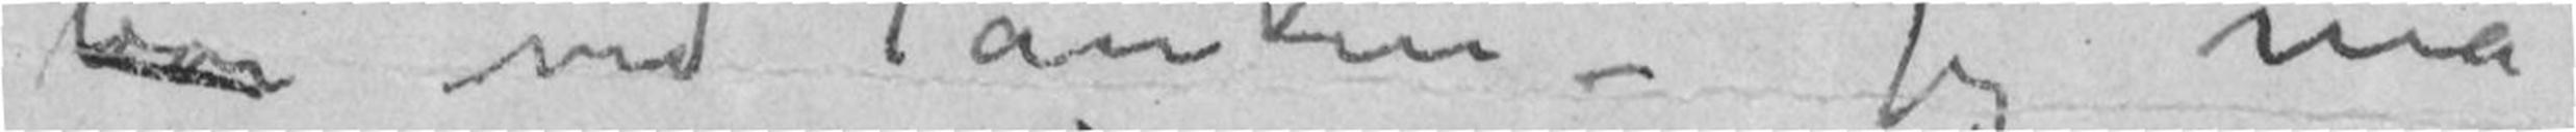ved Tanken – Jeg må
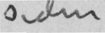siden
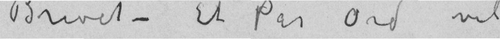Brevet – Et par Ord vil
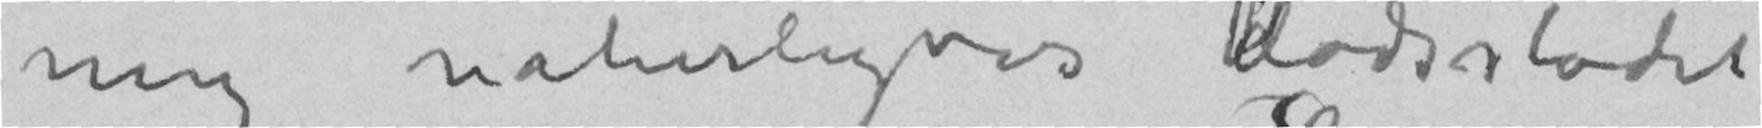mig naturligvis Dødsstødet
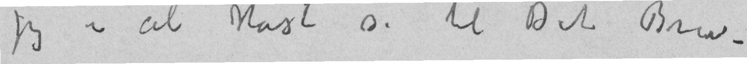jeg i al Hast si til Dit Brev –
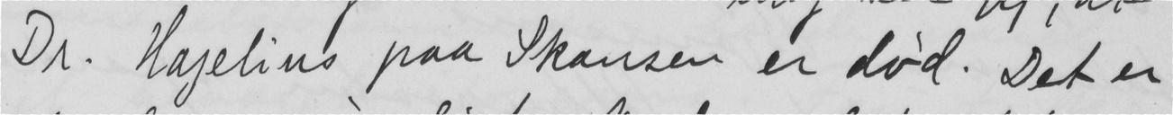Dr. Hagelius paa Skansen er død. Det er
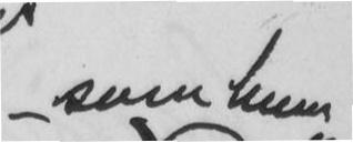som hun
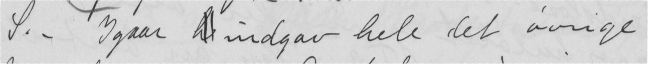S. - Igaar  indgav hele det ivrige
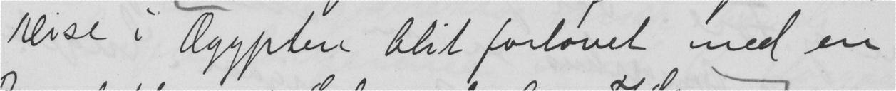reise i Ægypten blit forlovet med en
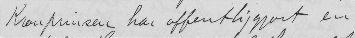Kronprinsen har affentliggjort en
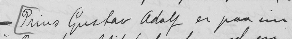- Prins Gustav Adolf er paa sin
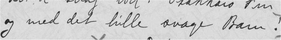og med det lille svage Barn.
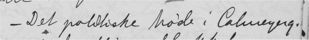- Det politiske Møde i Calmeyerg.
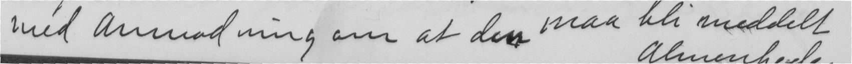med Anmodning om at den maa bli meddelt
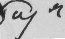og er
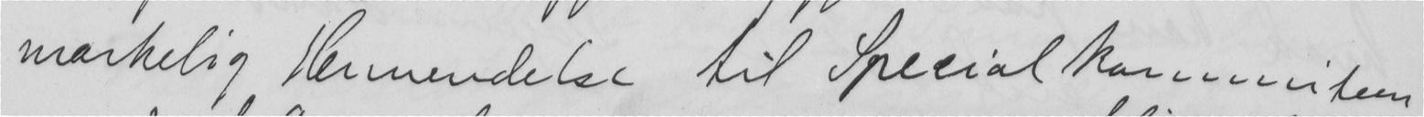mærkelig Hennendelse til Specialkommiteen
